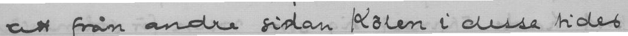att från andre sidan Kölen i disse tider
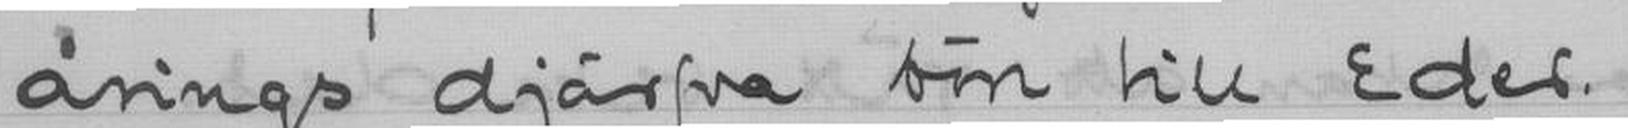årings djärfva bön till Eder.
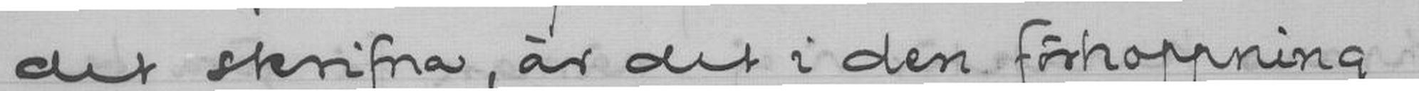det skrifna, är det i den förhoppning
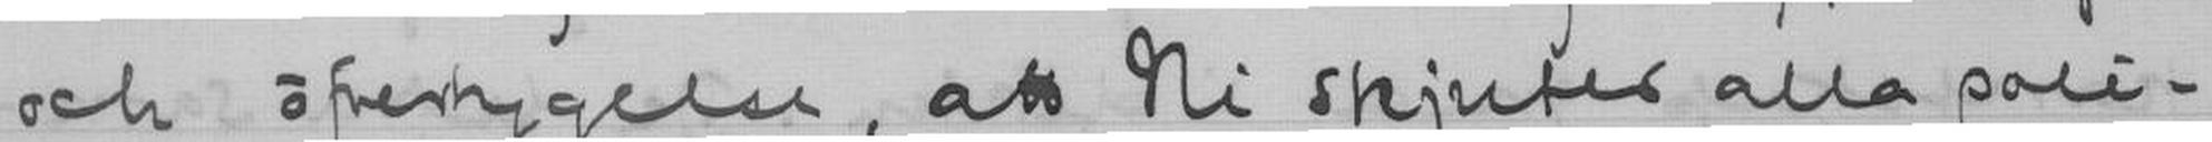och öfvertygelse, att Ni skjuter alla poli-
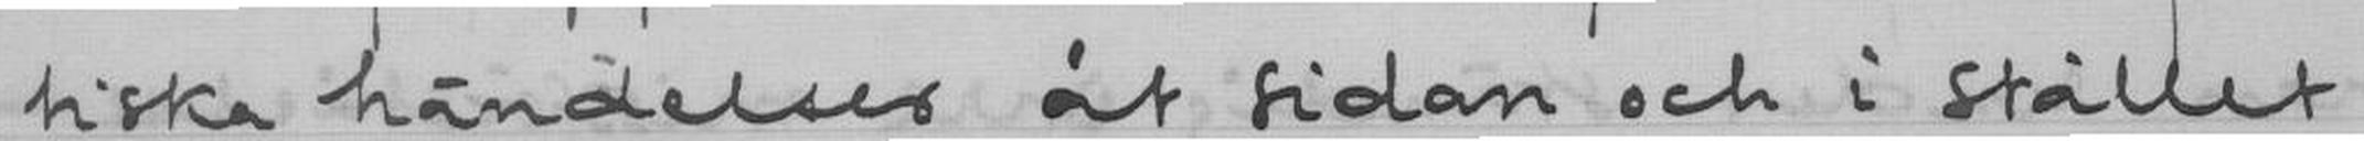tiska händelser åt sidan och i stället
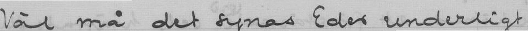Väl må det synas Eder underligt
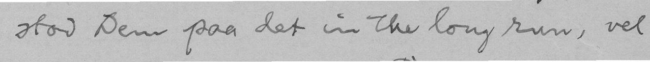stod Dem paa det in the long run, vel
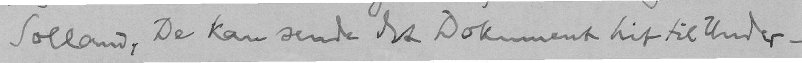Solland, De kan sende det Dokument hit til Under-
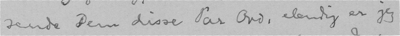sende Dem disse Par Ord, elendig er jeg
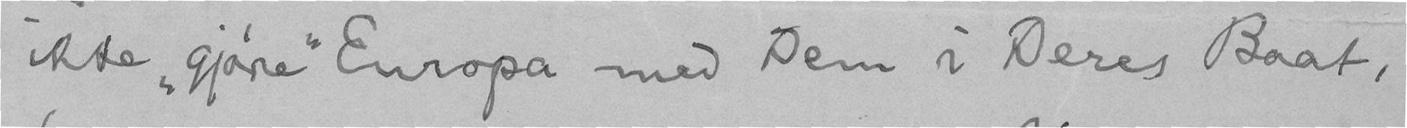ikke "gjøre" Europa med Dem i Deres Baat,
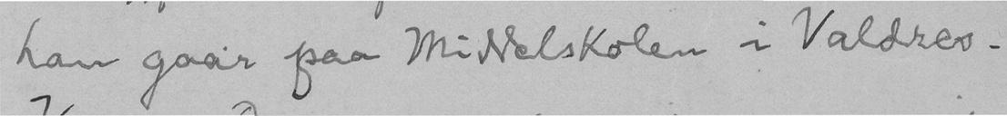han gaar paa Middelskolen i Valdres.
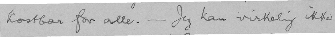kostbar for alle. - Jeg kan virkelig ikke
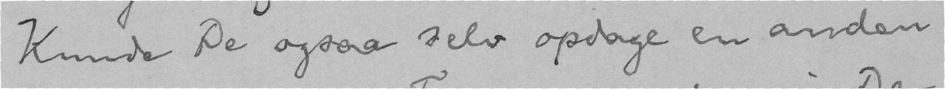Kunde De ogsaa selv opdage en anden
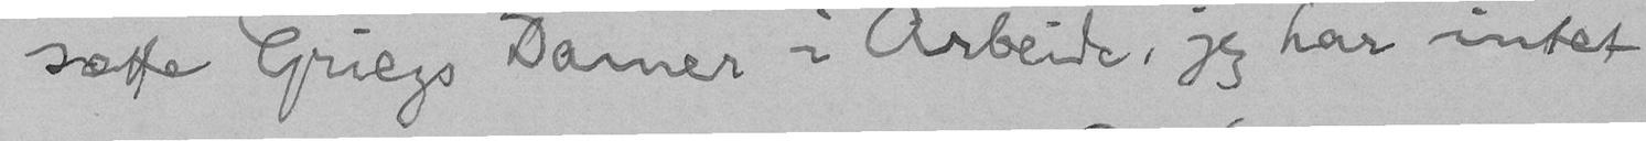sætte Griegs Damer i Arbeide, jeg har intet
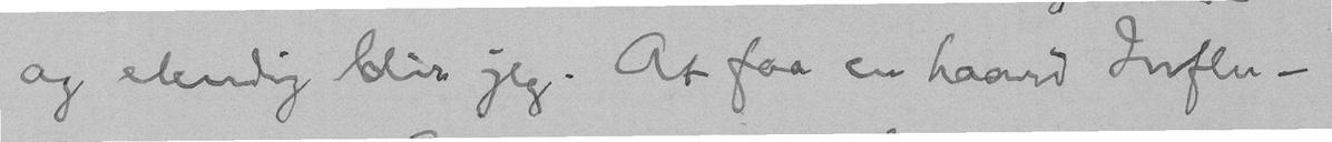og elendig blir jeg. At faa en haard Influ-
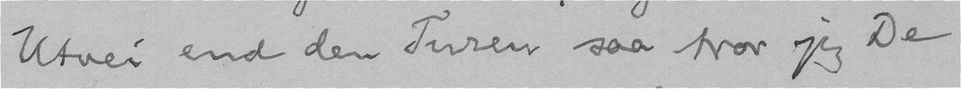Utvei end den Turen saa tror jeg De
